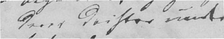deres Drifter under
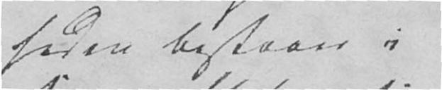heden bestaaer i
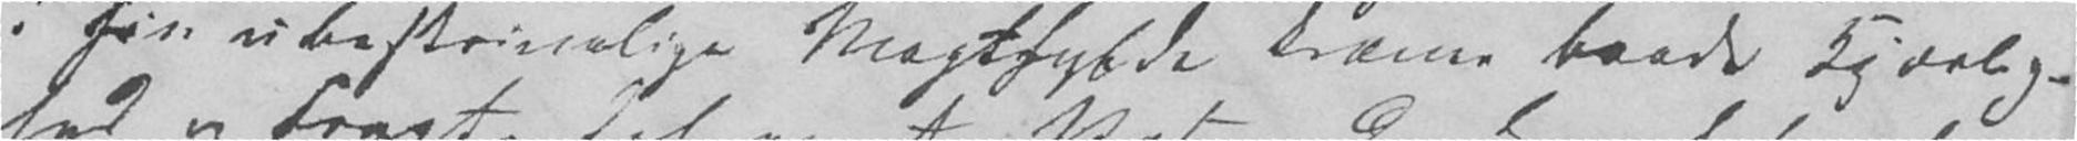i sin ubeskrivelige Magtfylde kræver baade Kjærlig-
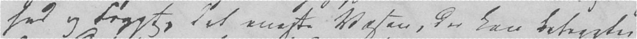hed og Frygt, det eneste Væsen, der kan betragtes
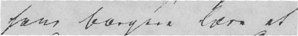hans Borgere lære at
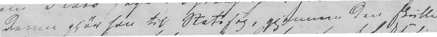Denne gjør han til Statssag, gjennem den skulle
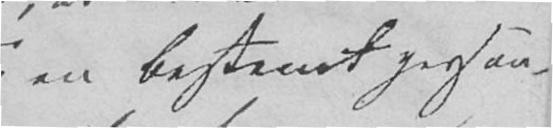en bestemt person-
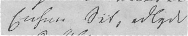Enhver Sit, adlyde
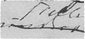X
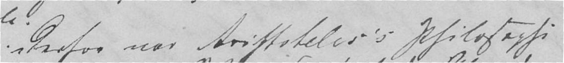Derfor var Aristoteles's Philosophi
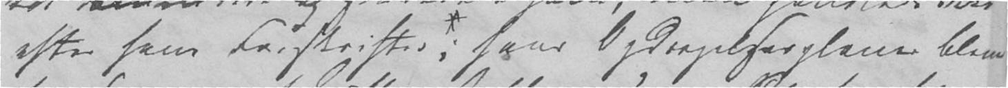efter hans Forskrifter, hans Opdragelsesplaner blev
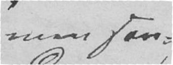men san-
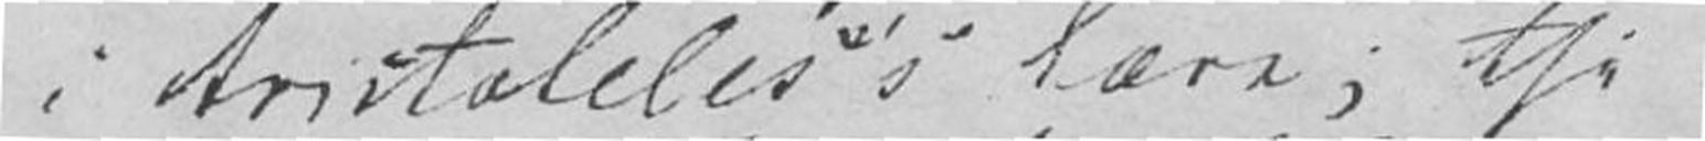i Aristoteles's Lære; thi
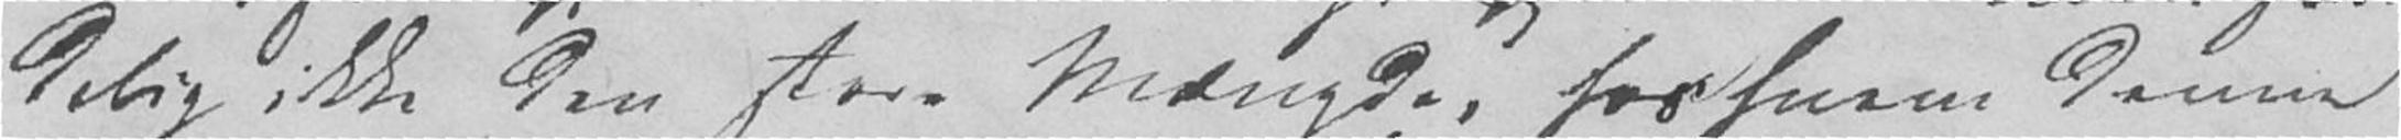delig ikke den store Mængde, hos hvem denne
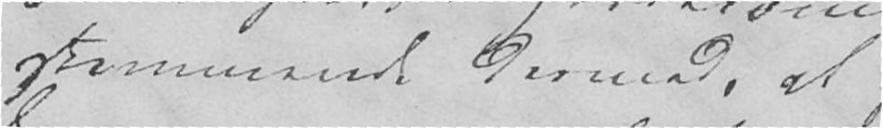stemmende dermed, at
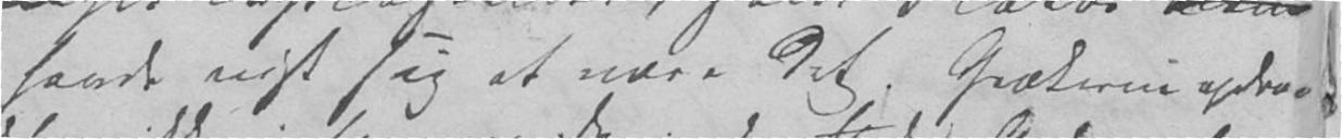havde vist sig at være det. Grækerne opdro-
